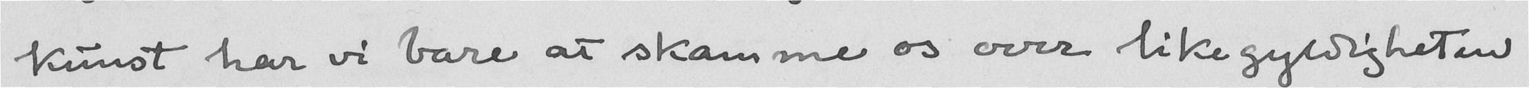kunst har vi bare at skamme os over likegyldigheten
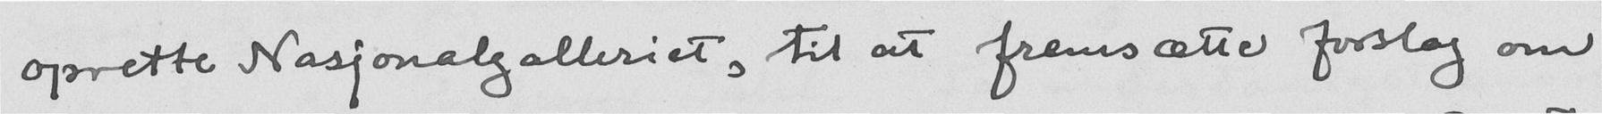oprette Nasjonalgalleriet, til at fremsætte forslag om
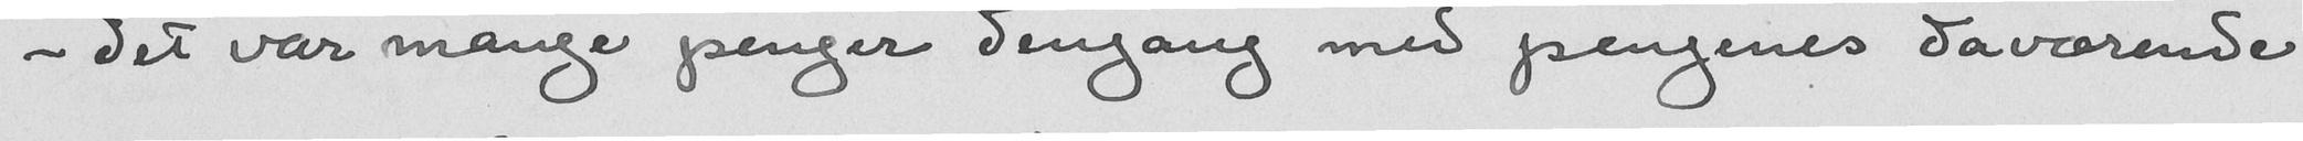- det var mange penger dengang med pengenes daværende
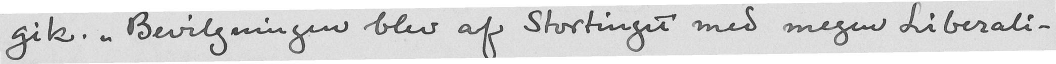gik. "Bevilgningen blev af Stortinget med megen Liberali-
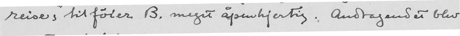reise," tilføier B. meget åpenhjertig. Andragendet blev
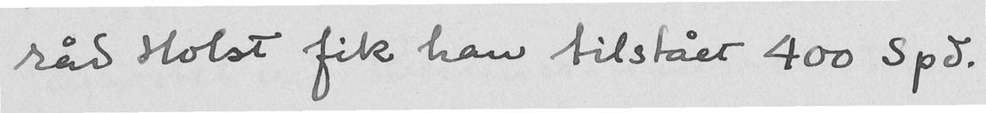råd Holst fik han tilstået 400 Spd.
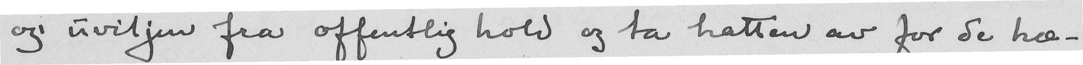og uviljen fra offentlig hold og ta hatten av for de hæ-
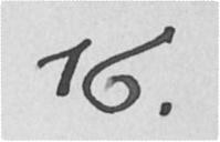16.
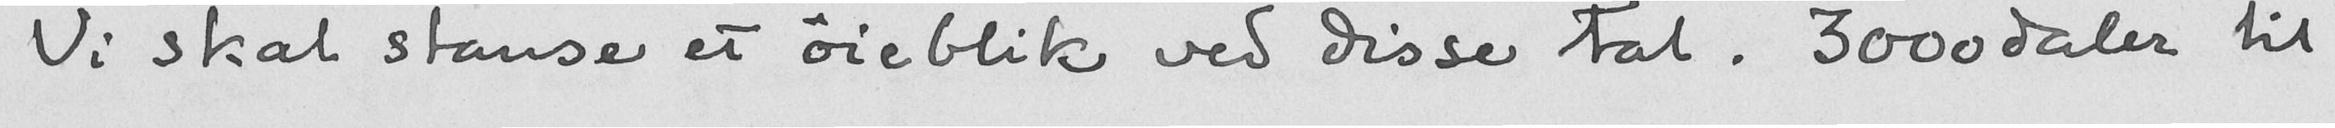Vi skal stanse et øieblik ved disse tal. 3000 daler til
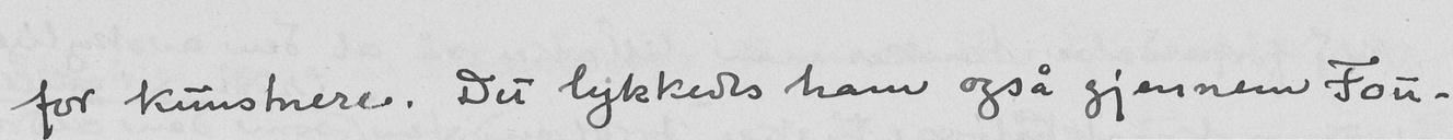for kunstnere. Det lykkedes ham også gjennem Fou-
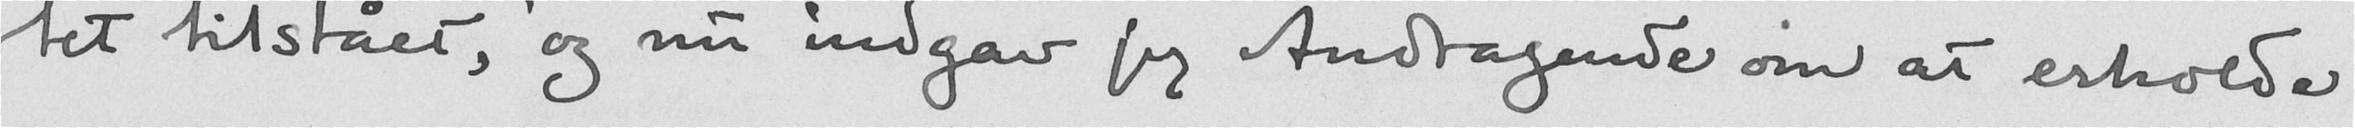tet tilstået," og nu indgav jeg Andtagende om at erholde
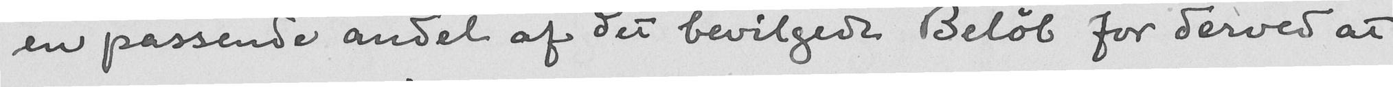en passende andel af det bevilgede Beløb for derved at
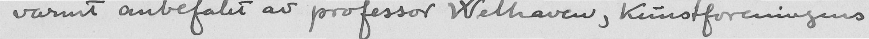varmt anbefalet av professor Welhaven, kunstforeningens
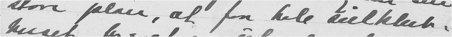store plan, at faa hele seilklub-
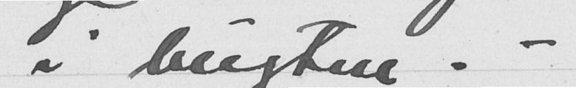i bugten. -
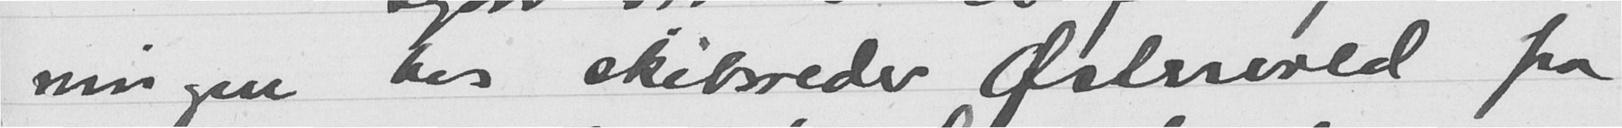ningen hos skibsreder Østervold fra
